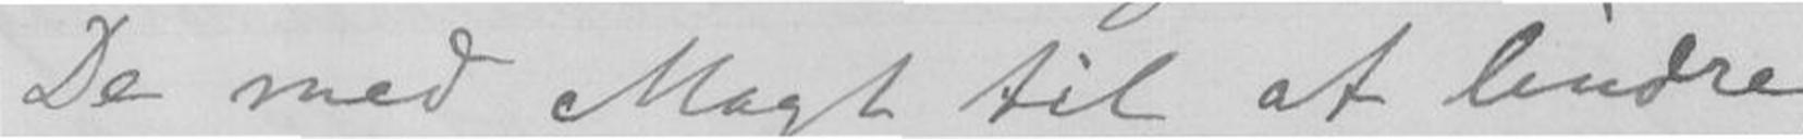De med Magt til at lindre,
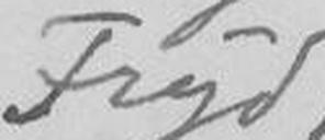Fryd,
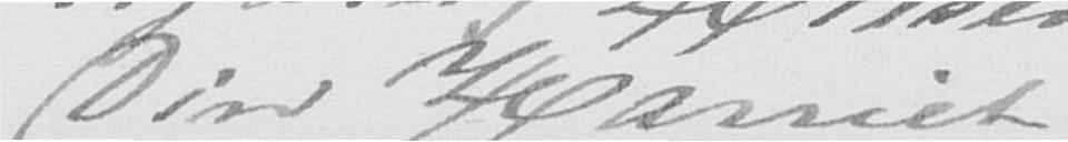Din Harriet
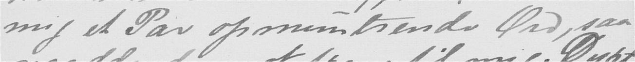mig et Par opmuntrende Ord, saa
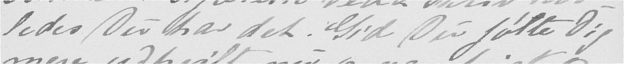ledes Du har det. Gid Du følte Dig
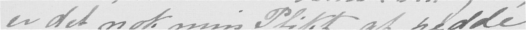er det nok min Plikt at redde
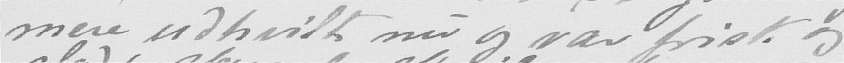mere udhvilt, nu og var frisk og
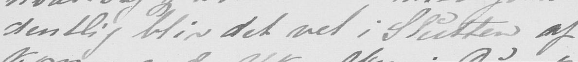dentlig blir det vel i Slutten af
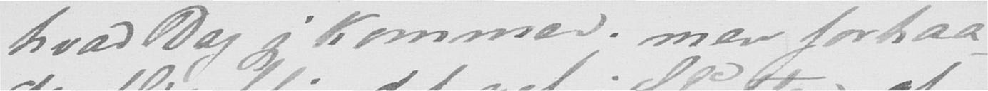hvad Dag jeg kommer - men forhaa-
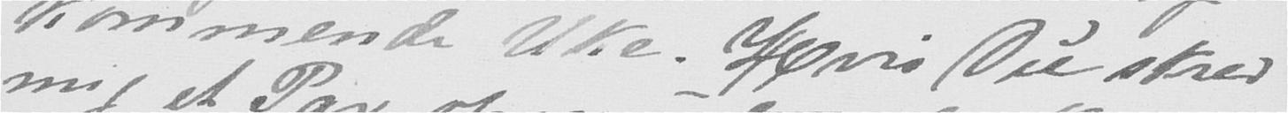kommende Uke. Hvis Du skrev
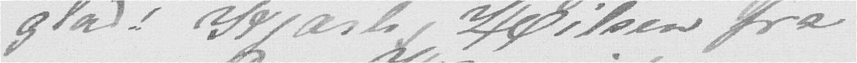glad! Kjærlig Hilsen fra
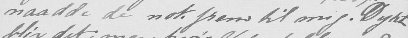naadde de nok frem til mig. Dyrt
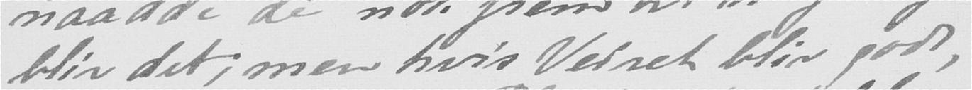blir det; men hvis Veiret blir godt,
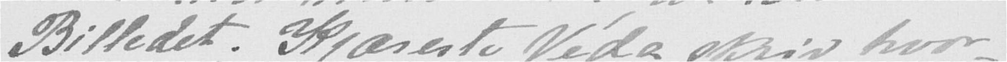Billedet. Kjæreste Veda skriv hvor-
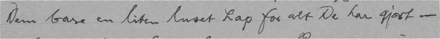Dem bare en liten luset Lap for alt De har gjort -
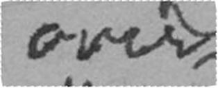over
